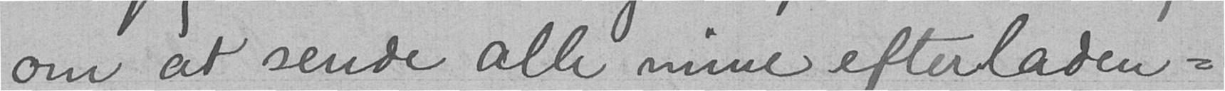om at sende alle mine efterladen-
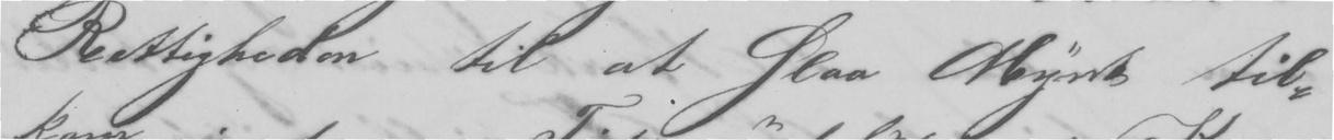Rettigheden til at Slaa Mynt til-
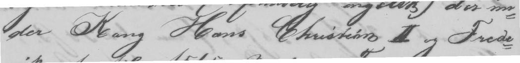der Kong Hans Christian II og Frede-
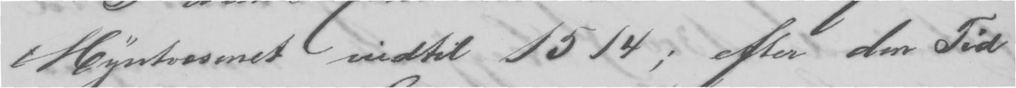Myntvæsenet indtil 1514, efter den Tid
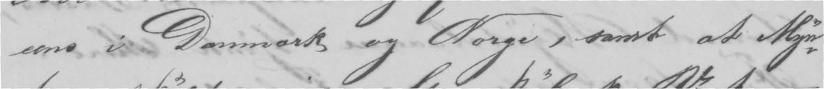ens i Dammark og Norge, samt at Myn-
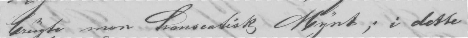brugte man hanseatisk Mynt; i dette
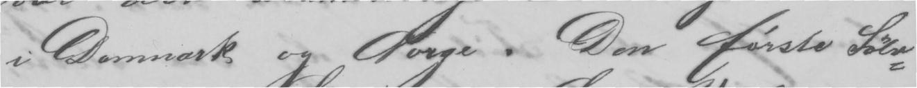i Danmark og Norge. Den første Sølv-
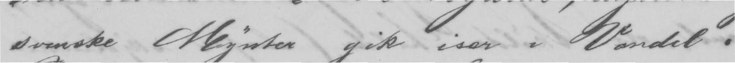svenske Mynter gik især i Vandel.
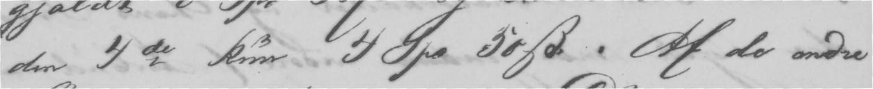den 4de kun 4 Spe 30 ẞ. Af de andre
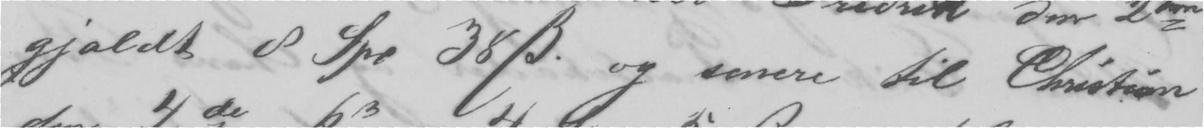gjaldt 8 Spe 38 ẞ. og senere til Christian
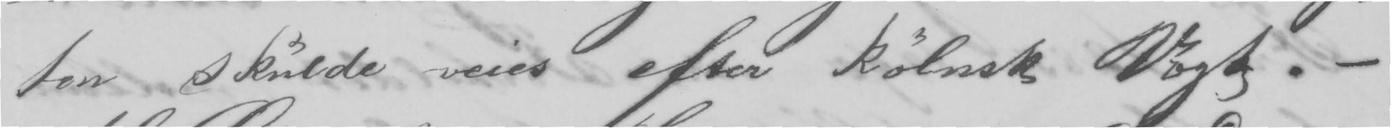ten skulde veies efter kølnsk Vægt. -
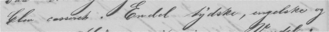blev casseret. Endel tydske, engelske og
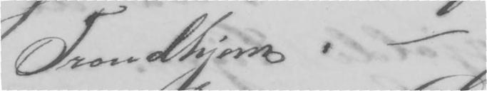Trondhjem. -
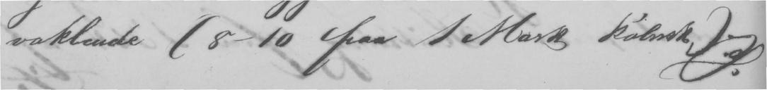vaklende (8-10 paa 1 Mark kølnsk).
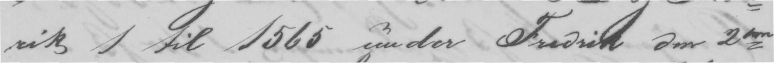rik 1 til 1565 under Fredrik den 2de
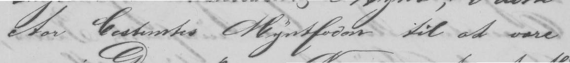Aar bestemtes Myntfoden til at være
